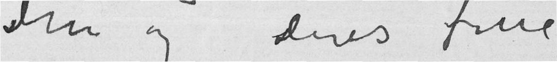dem og deres frue
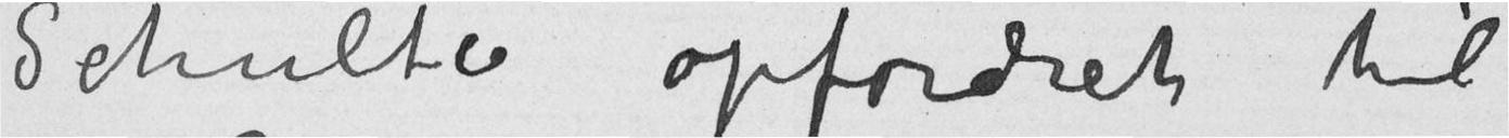Schulte opfordret til
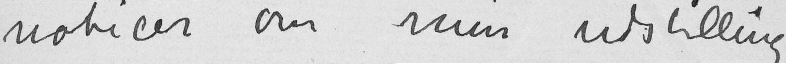noticer om min udstilling
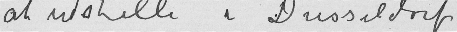at udstille i Düsseldorf
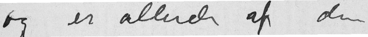og er allerede af den
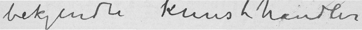bekjente kunsthandler
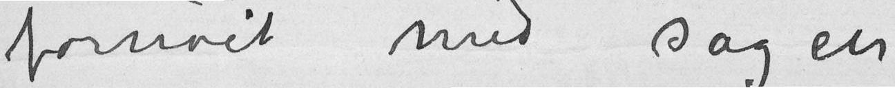fornøiet med sagen
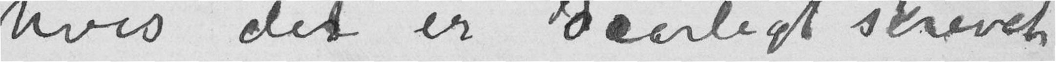hvis det er daarligt skrevet
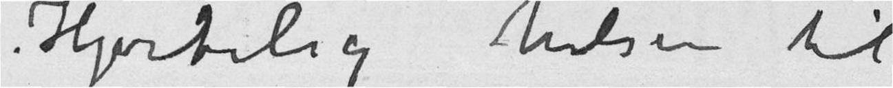Hjertelig hilsen til
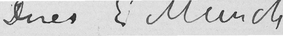Deres E Munch
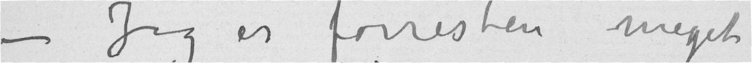– Jeg er forresten meget
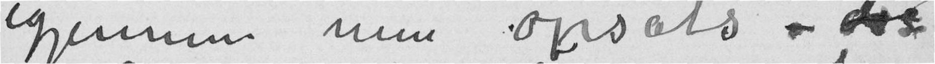gjennem min opsats –
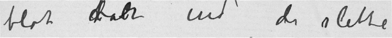blot tar ind de slette
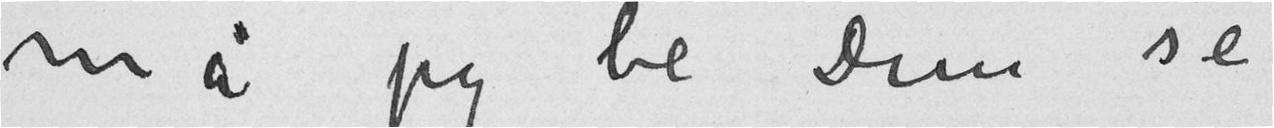må jeg be Dem se
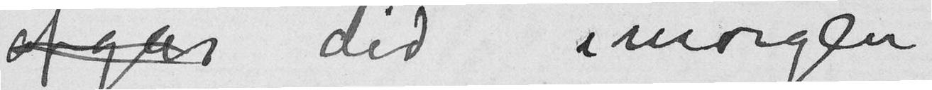afgår did imorgen
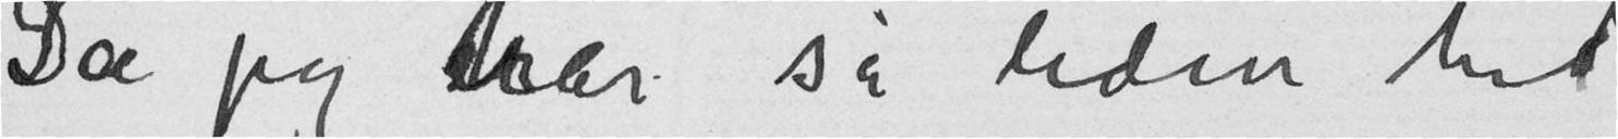Da jeg har så liden tid
